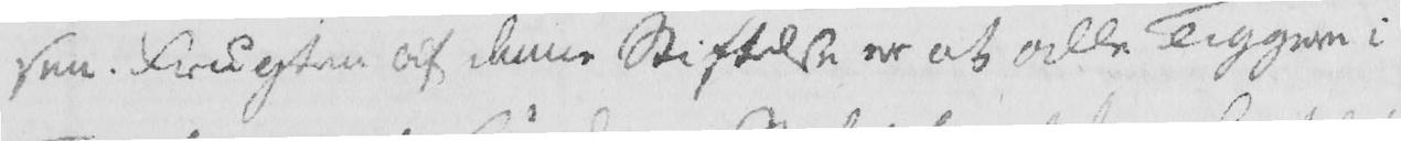sen. Frugten af denne Stiftelse er at alle Tiggere i
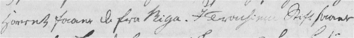Hørret faaer de fra Riga. I Tronhiem Stift saaer
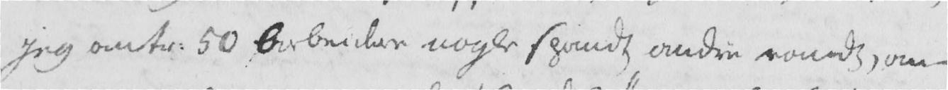jeg omtr. 50 Arbeidere nogle spandt andre randt, an-
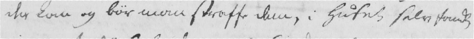der kan og bør man straffe dem, i Huset selv fandt
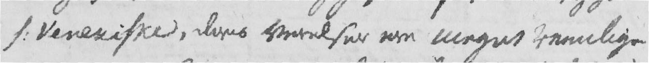/: Veneriske, deres værelser ere meget reenlige.
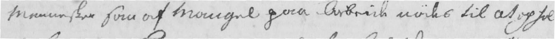Mennesker som af mangel paa Arbeide nødes til at ophol-
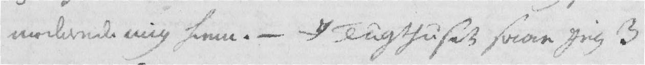mederede mig hiem. - I Tugthuset saae jeg 3
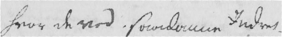hvor de ved saadanne Indret-
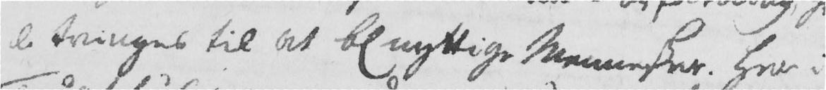de tvinges til at bl nyttige Mennesker. Her i
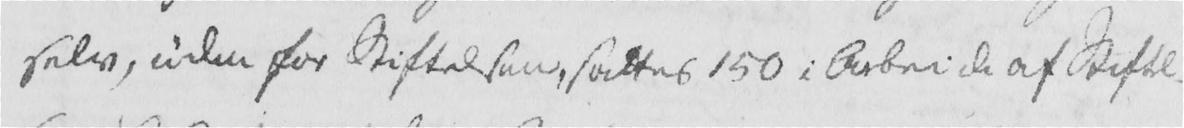selv, uden for Stiftelsen, sattes 150 i Arbeide af Stiftel-
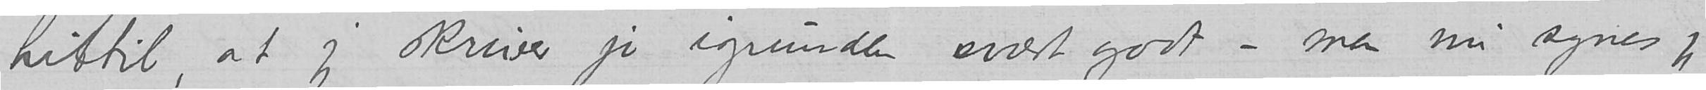hittil, at jeg skriver jou igrunden svært godt – men nu synes jeg
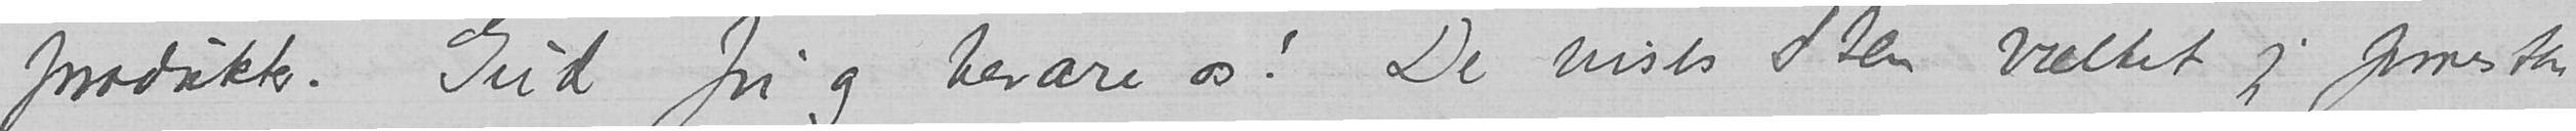produkter. Gud fri og bevare os! De vises Sten væltet jeg forresten
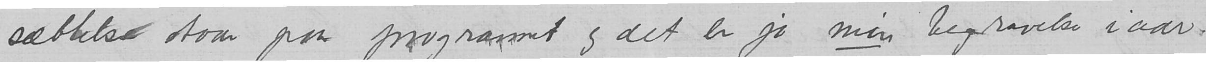sættelser staar paa programmet og det er jo min begravelse iaar.
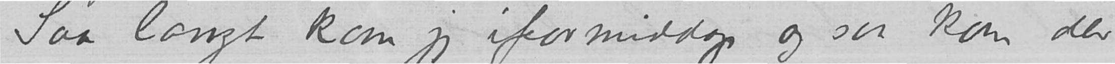-Saa langt kom jeg iformiddags og saa kom der
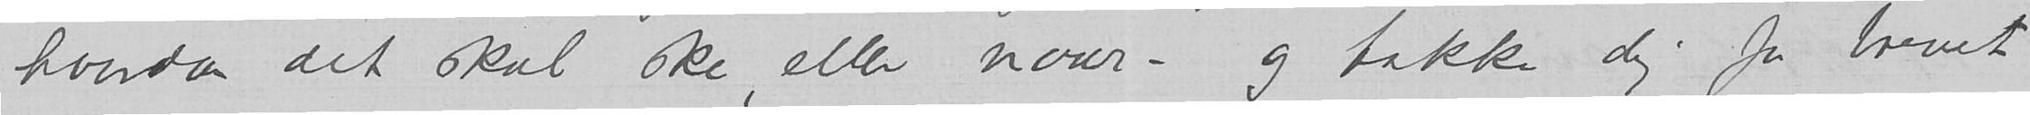hvordan det skal ske, eller naar – og takker dig for brevet
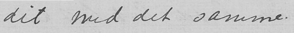dit med det samme.
